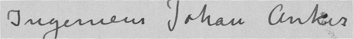Hr Ingenieur Johan Anker
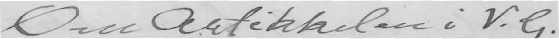Om Artikkelen i V.G.
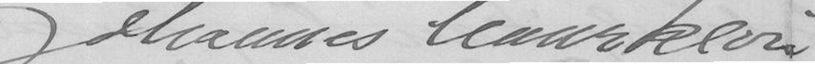Johannes Haarklau.
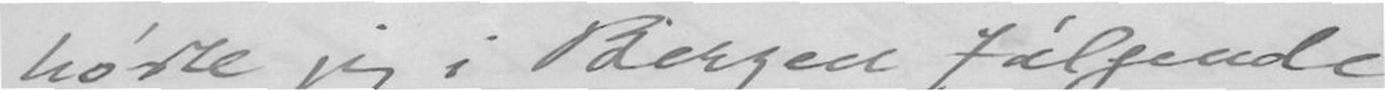hørdte jeg i Bergen følgende
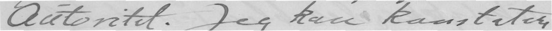Autoritet. Jeg kan konstatere
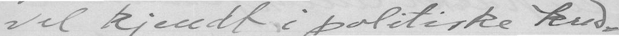vel kjendt i politiske kred-
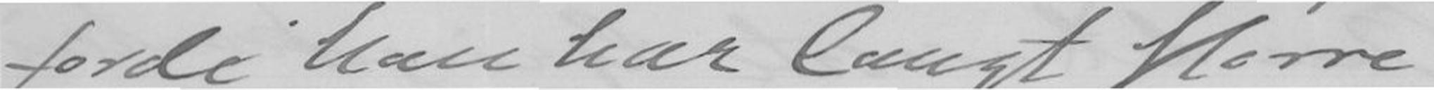fordi han har langt større
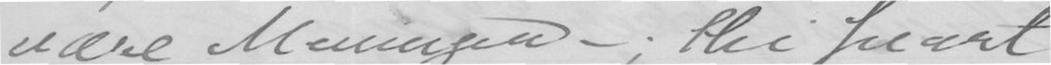være Meningen- ; thi snart
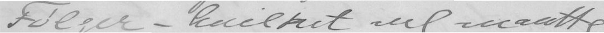Følger - hvilket vel maatte
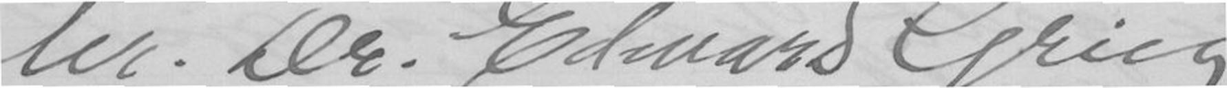Hr. Dr. Edward Grieg
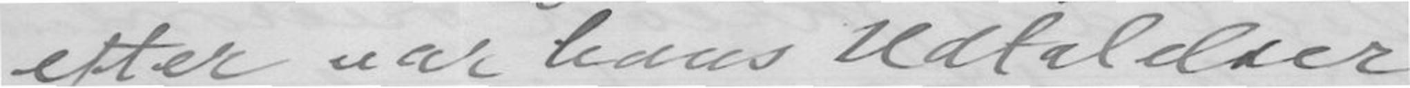efter var hans Udtalelser
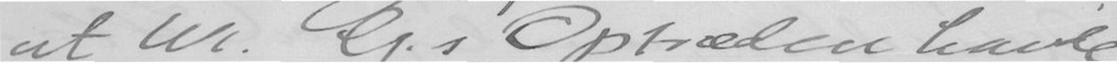at Hr. G.s Optræden havde
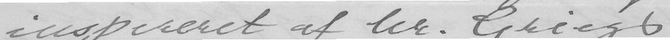inspireret af Hr. Grieg &
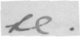se.
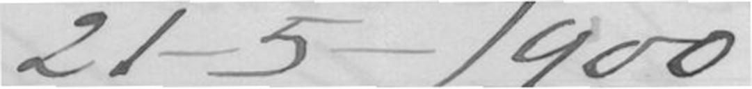21-5-1900
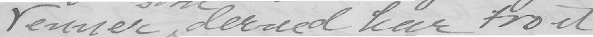Venner derved har troet
